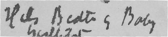Hils Beate og Baby
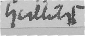hjerteligst
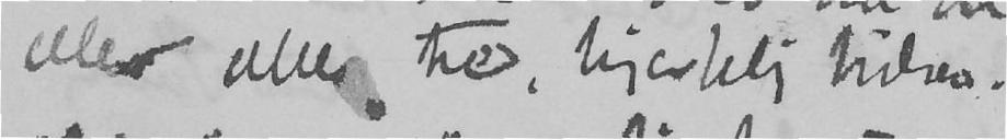eller alle tre, hjertelig hilsen.
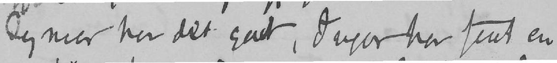Dagmar har det godt, Ingar har faat en
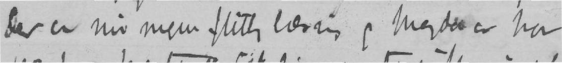Der er nu meget flittig læsing og Magda er hos
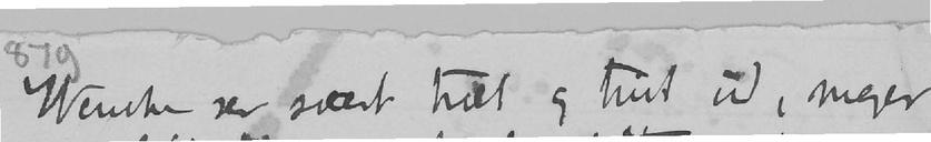Wenche ser svært træt og trist ud, mager
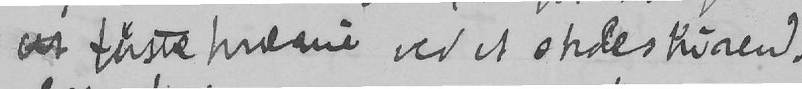at førstepræmie ved et skoleskirend.
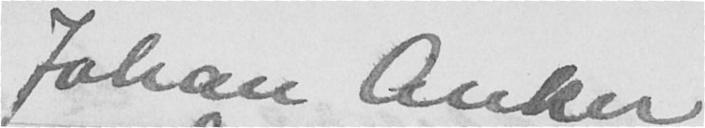Johan Anker
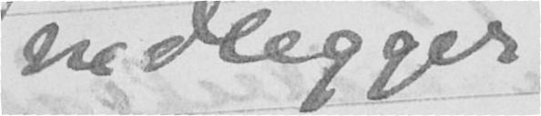nedlegges
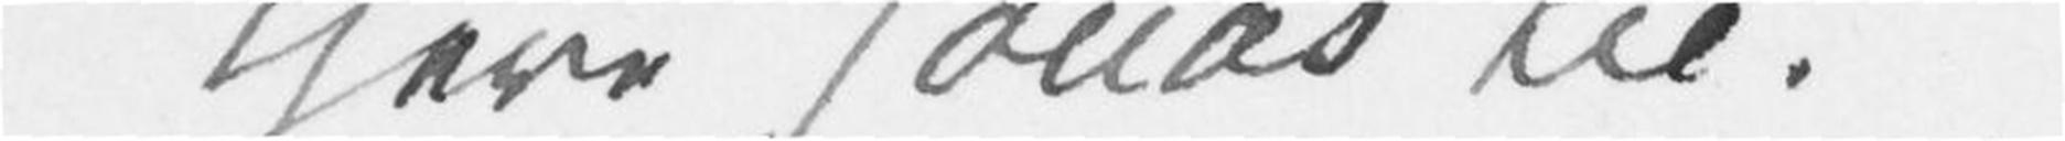Kjere Jonas Lie!
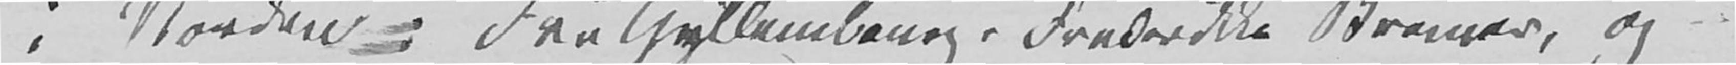i Norden: Fru Gyllembourg, Frederikka Bremer, og
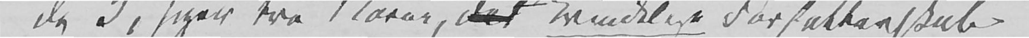de 3, siger tre Navne, det kvindelige Forfatterskab
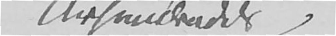Århundredets
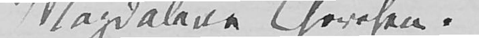Magdalene Thoresen.
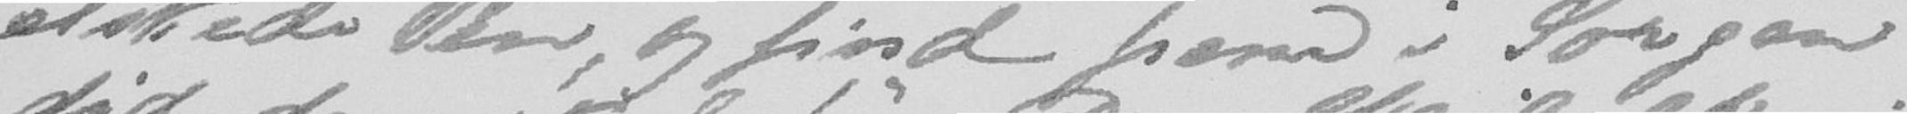elskede Ven, og find frem i Sorgen
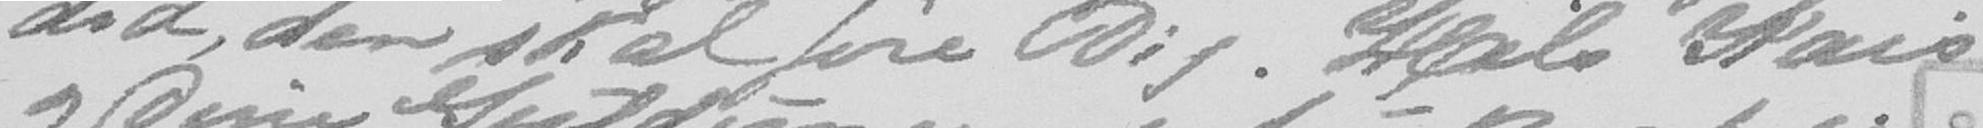did, den skal føre Dig. Hils Kais
